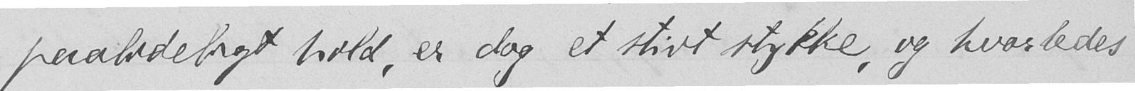paalideligt hold, er dog et stivt stykke, og hvorledes
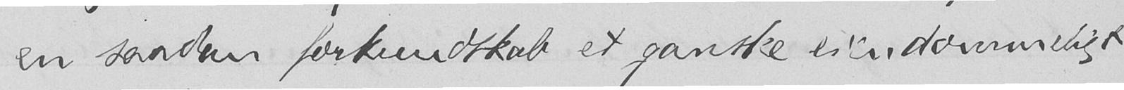en saadan forkundskab et ganske eiendommeligt
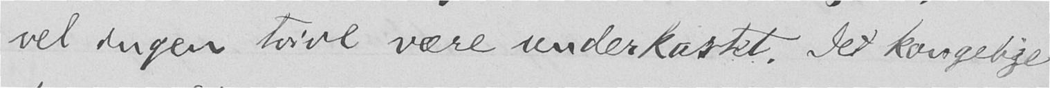vel ingen tvivl være underkastet. Det kongelige
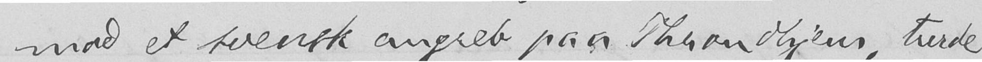mod et svensk angreb paa Throndhjem, turde
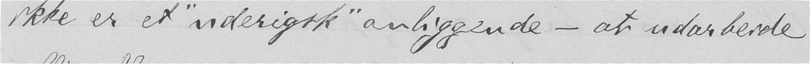ikke er et "uderigsk" anliggende - at udarbeide
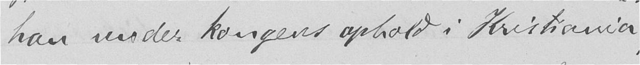han under kongens ophold i Kristiania
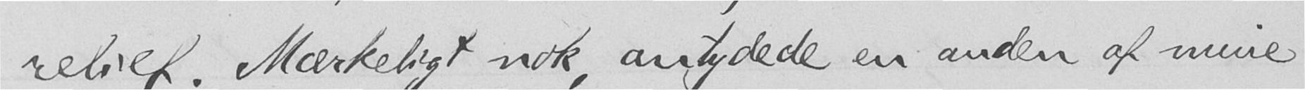relief. Mærkeligt nok, antydede en anden af mine
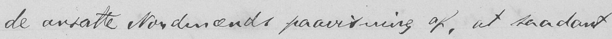de ansatte Nordmænds paavisning af, at saadant
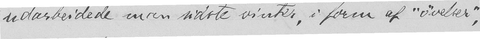udarbeidede man sidste vinter, i form af "øvelser",
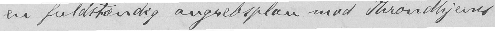en fuldstændig angrebsplan mod Throndhjems
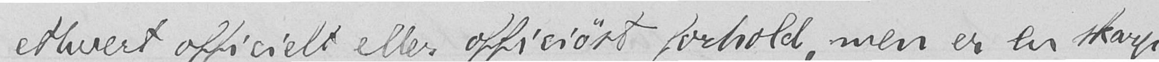ethvert officielt eller officiøst forhold - men er en skarp
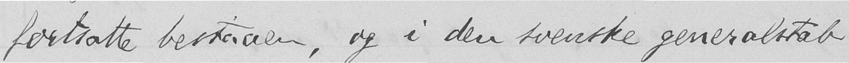fortsatte bestaaen, og i den svenske generalstab
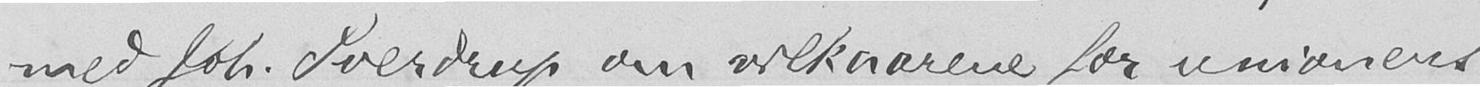med Joh. Sverdrup om vilkaarene for unionens
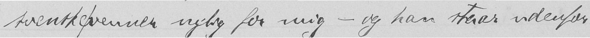svenske venner nylig for mig - og han staar udenfor
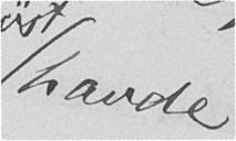havde
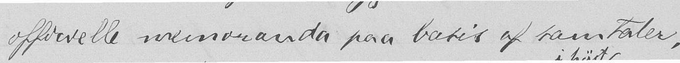officielle memoranda paa basis af samtaler,
